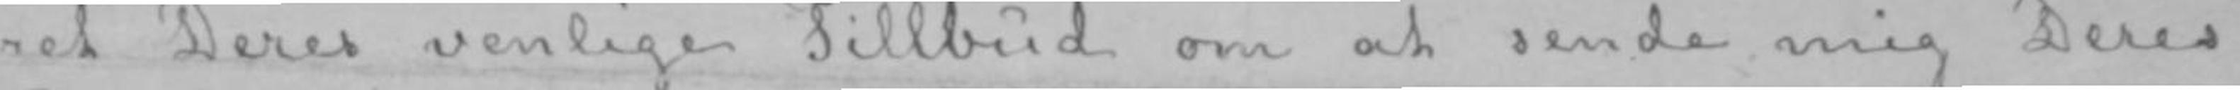ret Deres venlige Tillbud om at sende mig Deres
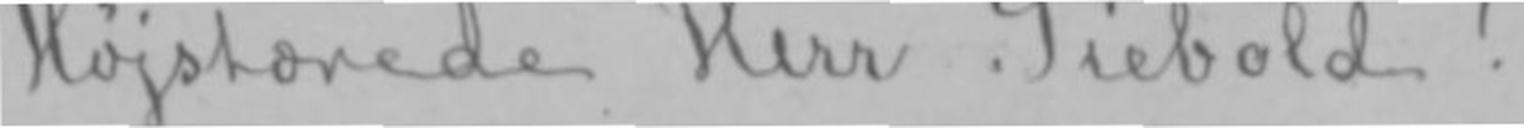Højstærede Herr Siebold!
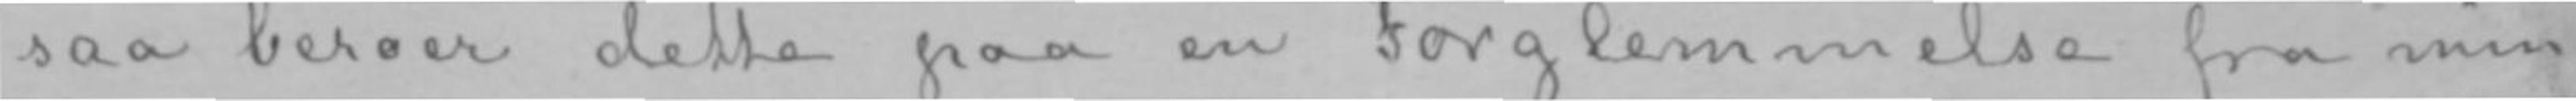saa beroer dette paa en Forglemmelse fra min
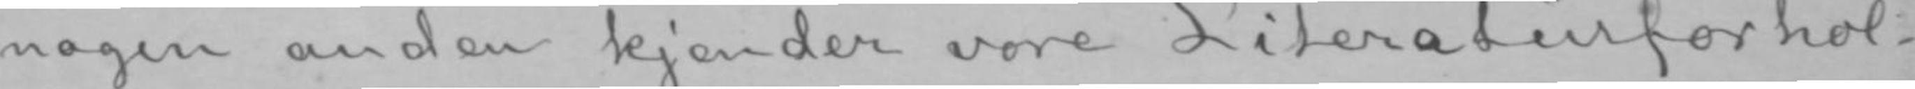nogen anden kjender vore Literaturforhol-
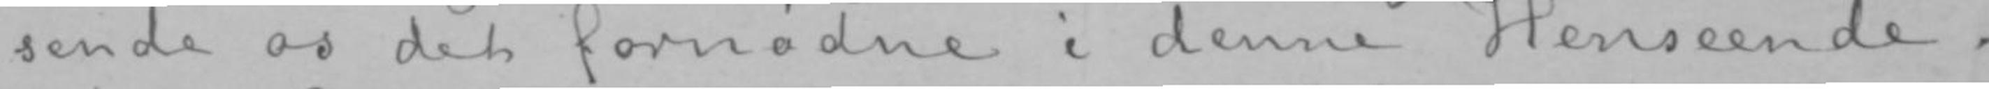sende os det fornødne i denne Henseende.
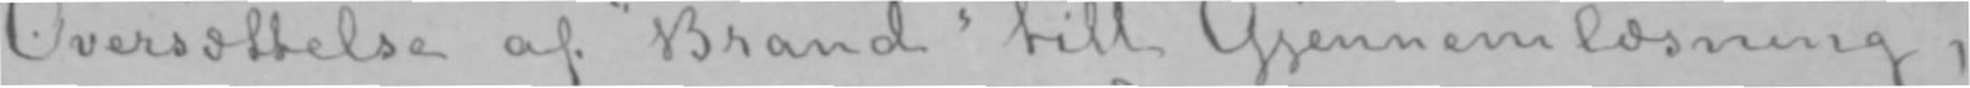Oversættelse af «Brand» till Gjennemlæsning,
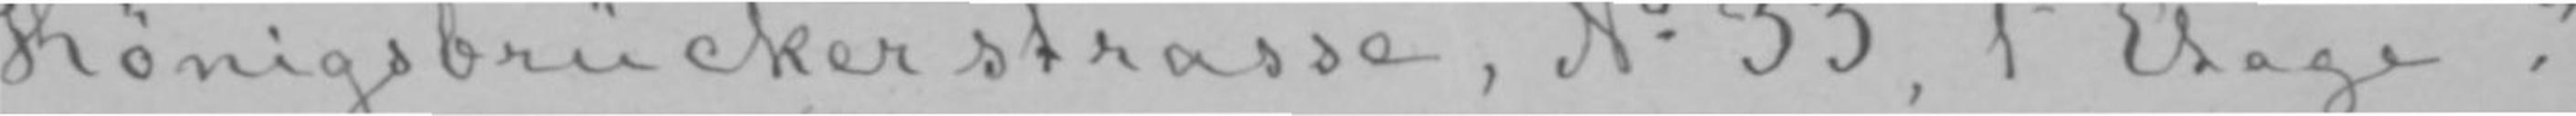«Königsbrückerstrasse, No 33, 1te Etage.»
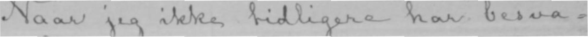Naar jeg ikke tidligere har besva-
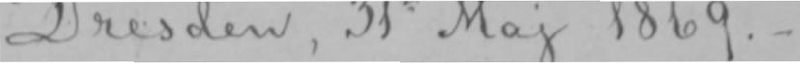Dresden, 31te Maj 1869.-
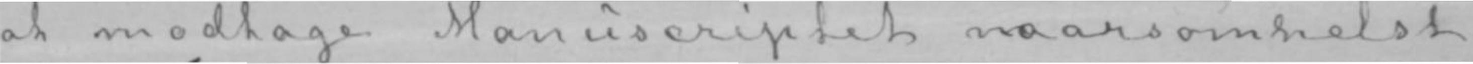at modtage Manuscriptet naarsomhelst
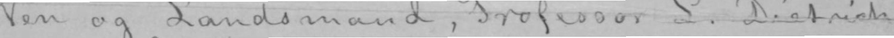Ven og Landsmand, Professor L. Dietrich-
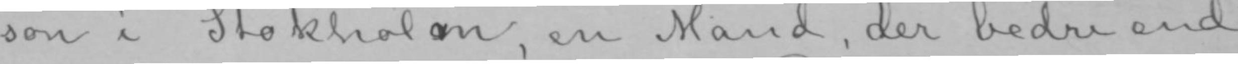son i Stokholm, en Mand, der bedre end
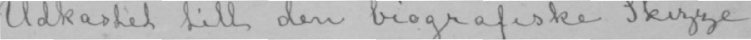Udkastet till den biografiske Skizze
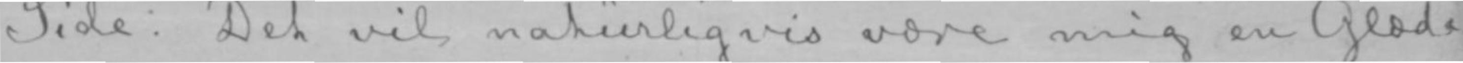Side. Det vil naturligvis være mig en Glæde
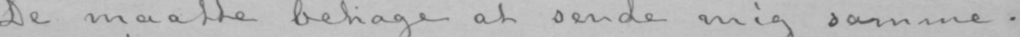De maatte behage at sende mig samme.
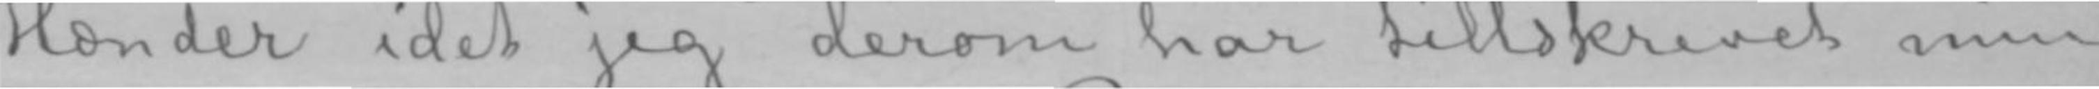Hænder idet jeg derom har tillskrevet min
